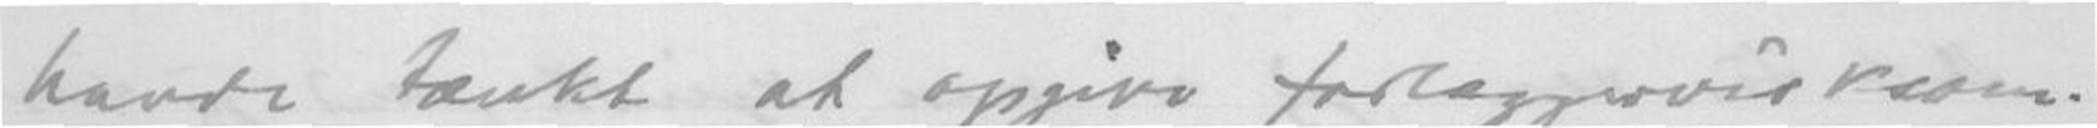havde tænkt at opgive forlæggervirksom-
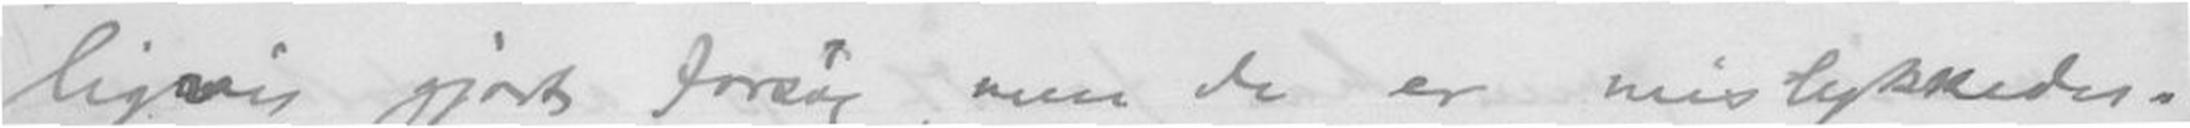ligvis gjort forsøg, men de er mislykkedes.
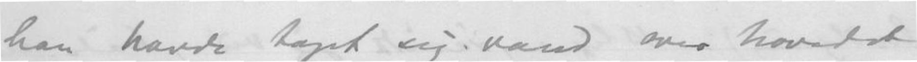han havde taget sig vand over hovedet
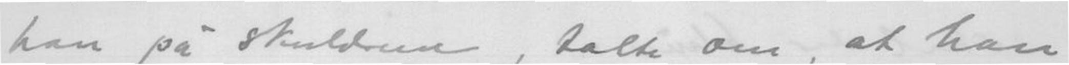han på skulderne, talte om at han
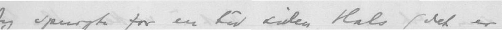Jeg spurgete for en tid siden Hals (det er,
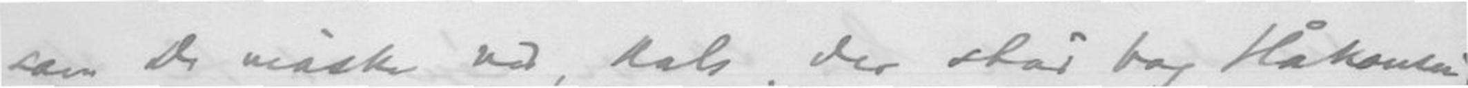som De måske ved, Hals, der står bag Håkonsen)
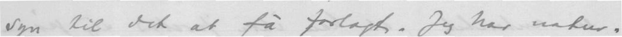syn til det at få forlagt. Jeg har natur-
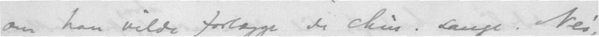om han vilde forlægge de chin. sange. Nei,
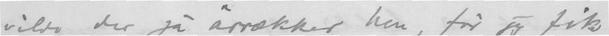vilde der gå årrækker hen, før jeg fik
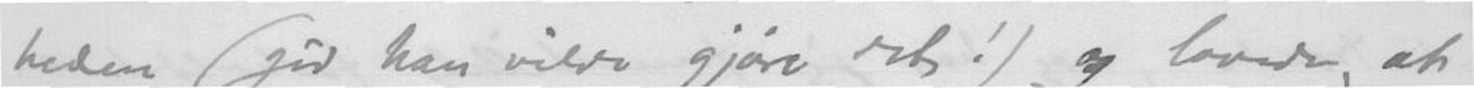heden (gid han vilde gjøre det!) og lovede, at
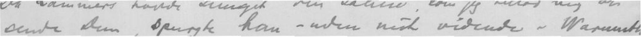sende Dem, spurte han - uden mit vidende - Warmuth,
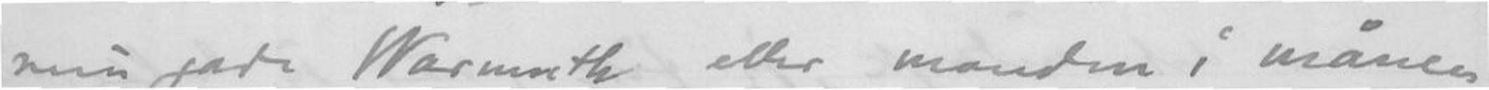min gode Warmuth eller manden i månen
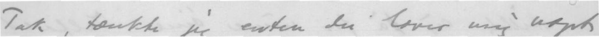Tak, tænkte jeg enten du lover mig noget
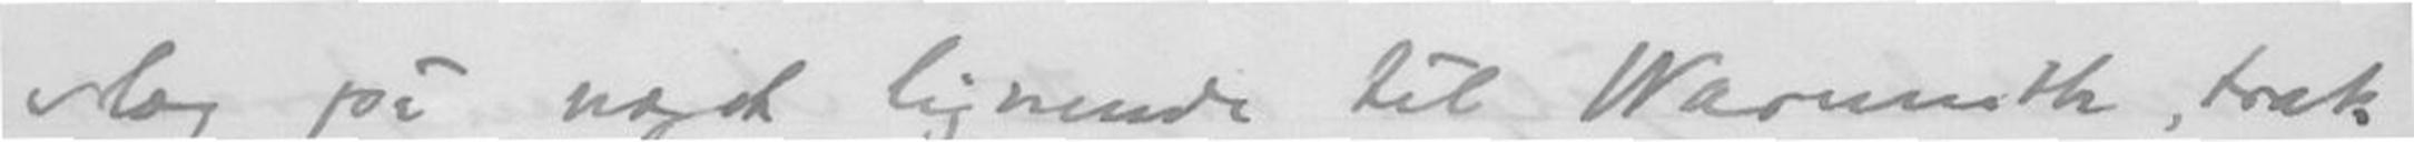slog på noget lignende til Warmuth, trak
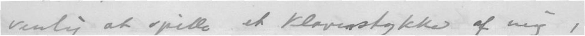venlig at spille et klaverstykke af mig,
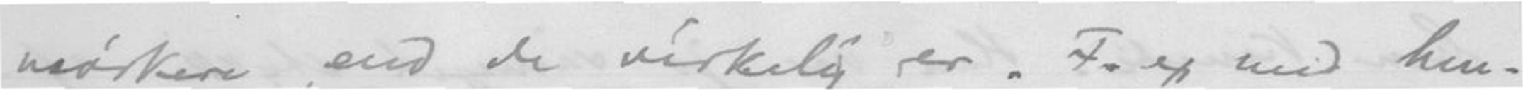mørkere, end de virkelig er. F.ex med hen-
